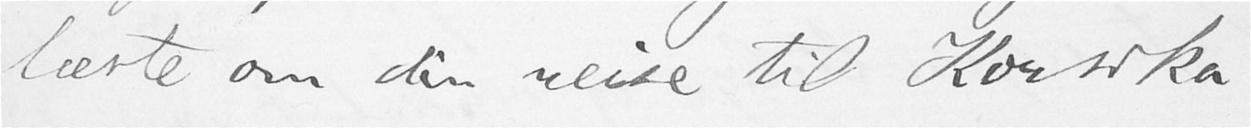læste om din reise til Korsika.
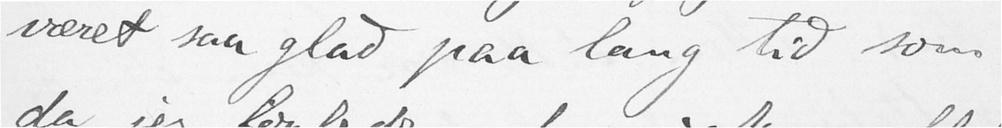været saa glad paa lang tid som
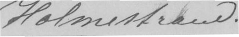Holmestrand.
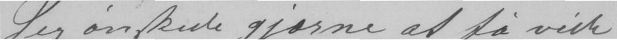Jeg ønskede gjærne at få vide
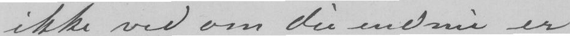ikke ved om du endnu er
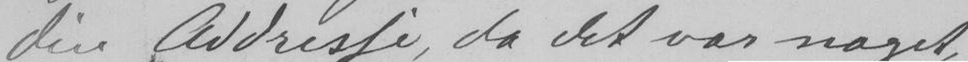din Addresse, da det var noget,
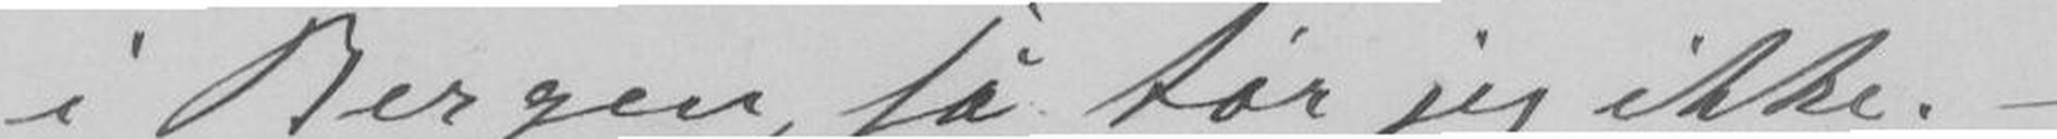i Bergen, så tør jeg ikke. -
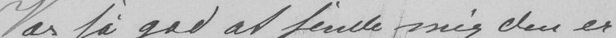Vær så god at sende mig den er
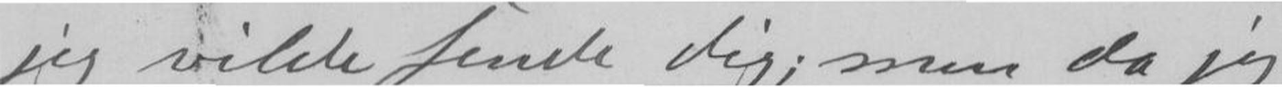jeg vilde sende dig; men da jeg
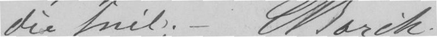du snil. -CBorch
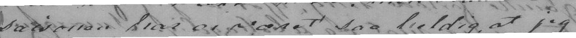saisonen har ei været saa heldig at jeg
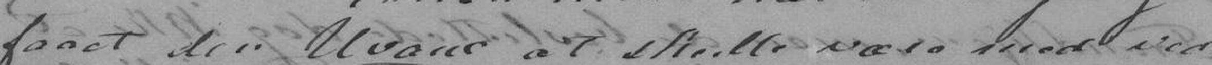faaet den Uvane at skulle være med ved
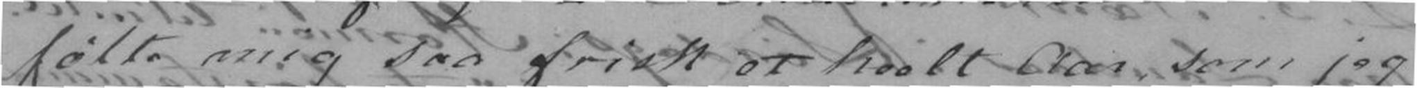følte mig saa frisk et heelt aar, som jeg
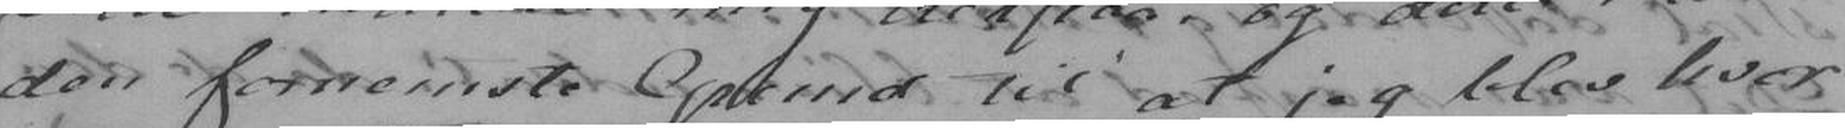den fornemste Grund til at jeg blev hvor
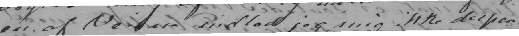en af Veiene indlod jeg mig ikke derpaa.
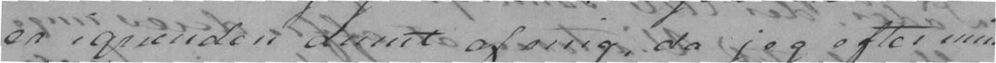er igrunden dumt af mig, da jeg efter min
